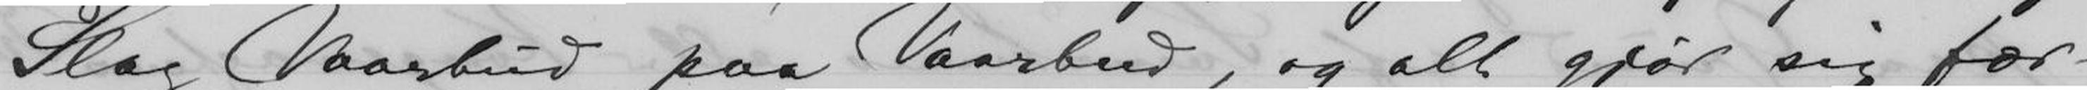Slag Vaarbud paa Vaarbud, og alt gjør sig fær-
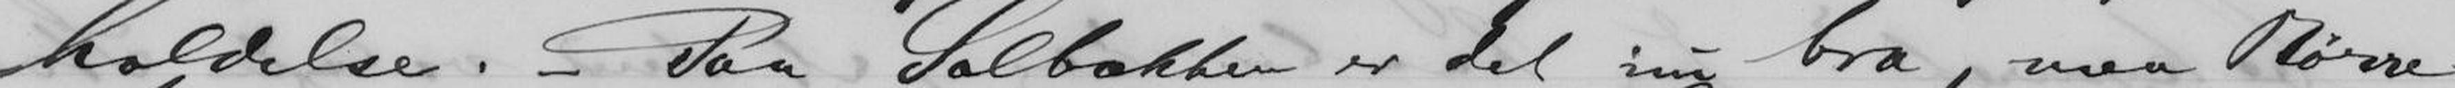holdelse. - Paa Solbakken er det nu bra, men Børre
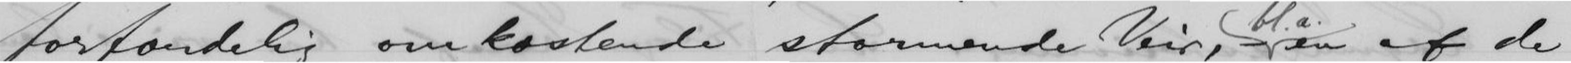forfærdelig omkastende stormende Veir, bl.a. en af de
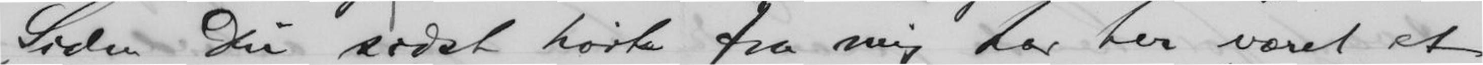Siden Du sidst hørte fra mig har her været et
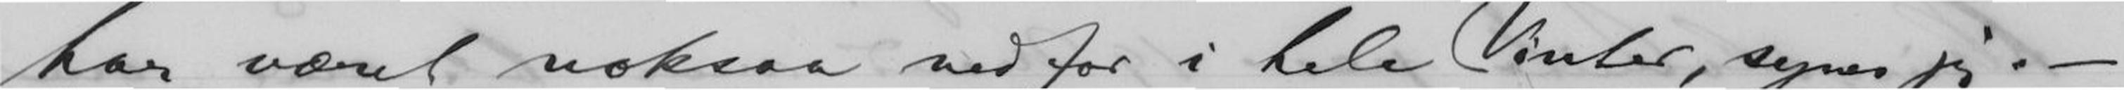har været noksaa nedfor i hele Vinter, synes jeg. -
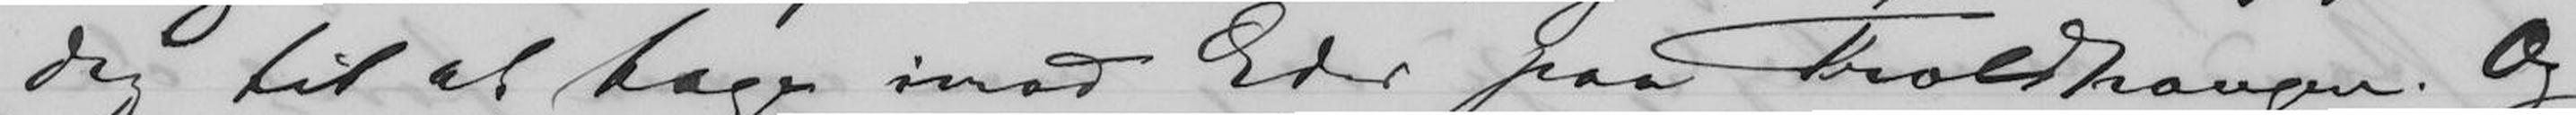dig til at tage imod Eder paa Troldhaugen. Og
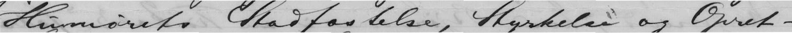Humørets Stadfæstelse, Styrkelse og Opret-
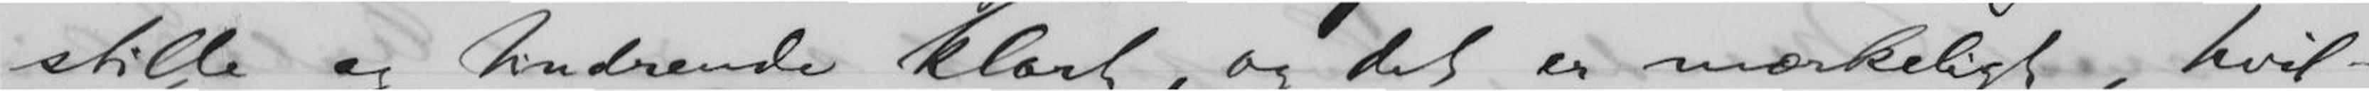stille og tindrende klart, og det er mærkeligt, hvil-
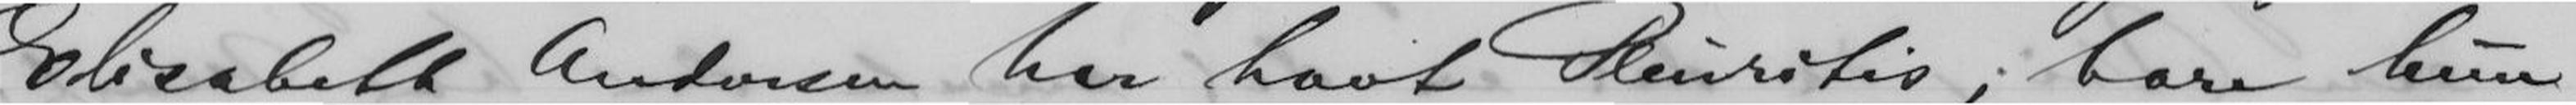Elisabeth Andersen har havt Pleuritis; bare hun
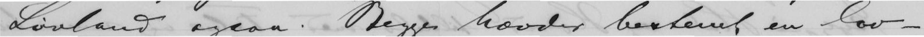Løvland ogsaa. Begge hævder bestemt en lov-
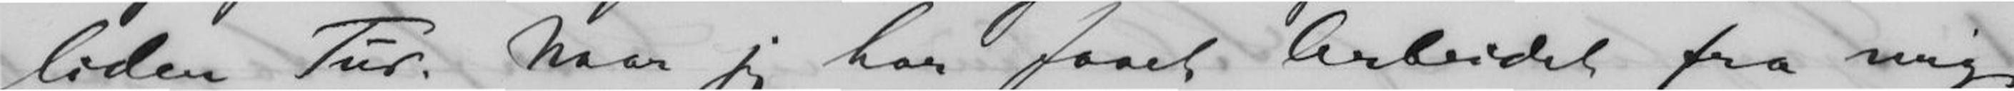liden Tur. Naar jeg har faaet Arbeidet fra mig,
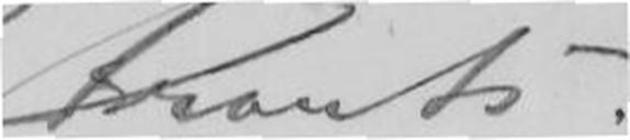Frants.
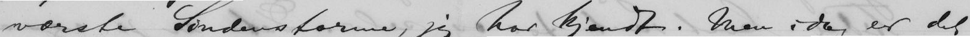værste Søndenstorme, jeg har kjendt. Men idag er det
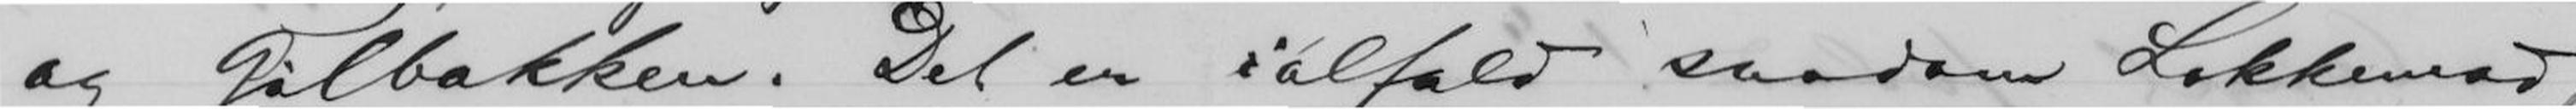og Gilbakken. Det er ialtfald saadan Lokkemad,
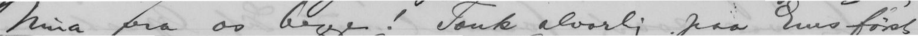Nina fra os begge! Tænk alvorlig paa Ems først
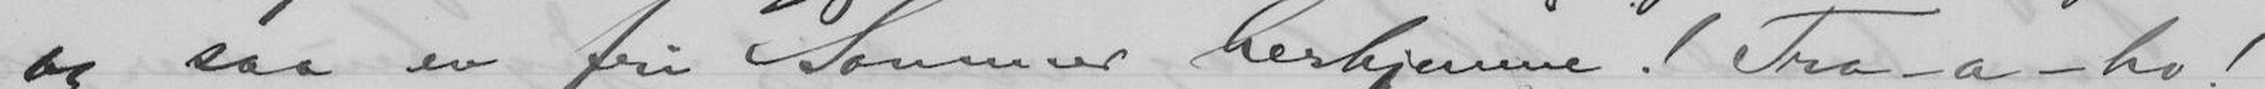og saa en fri Sommer herhjemme! Tra-a-ho!
